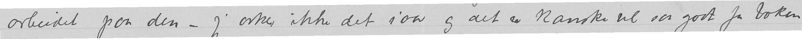arbeidet paa den – jeg orker ikke det iaar og det er kanske vel saa godt for boken
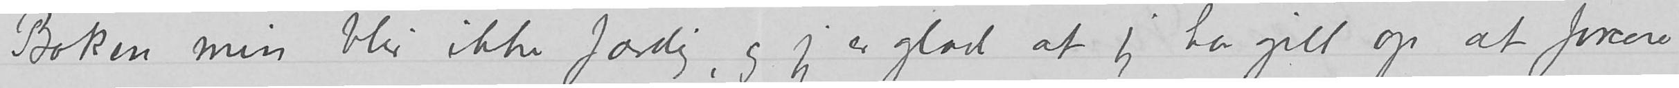Boken min blir ikke færdig, og jeg er glad at jeg har gitt op at forcere
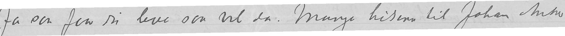Ja saa faar du leve saa vel da.  Mange hilsener til Johan Anker
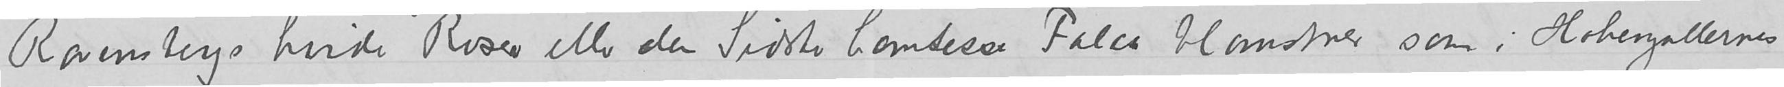Ravensburgs hvide Roser eller den Sidste Comtesse Falcs blomstrer som i Hohenzollernes
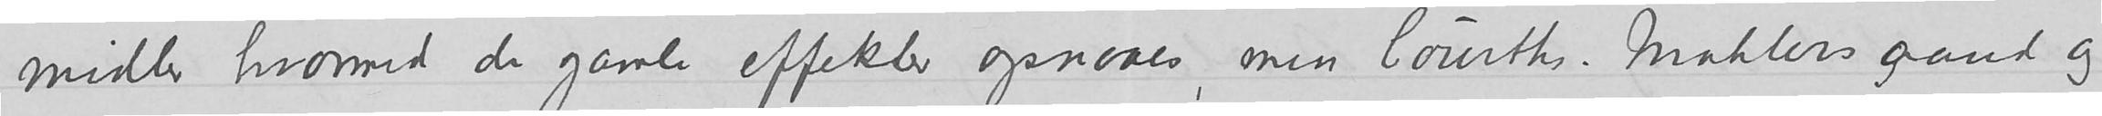midler hvormed de gamle effekter opnaaes, men Courths-Mahlers aand og
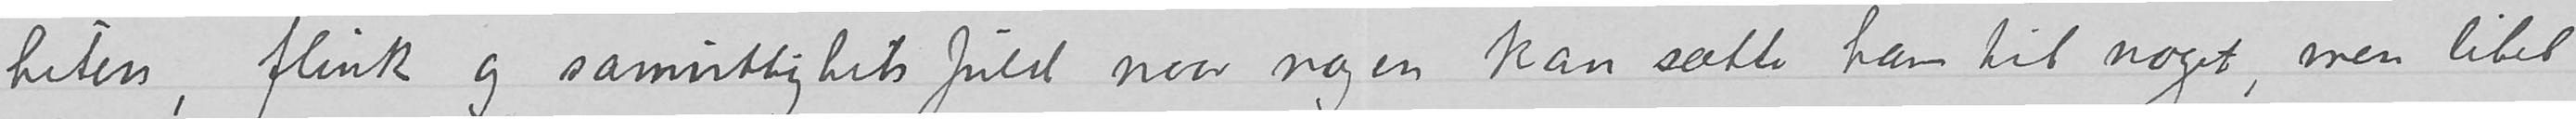heten, flink og samvittighetsfuld naar nogen kan sætte ham til noget, men litet
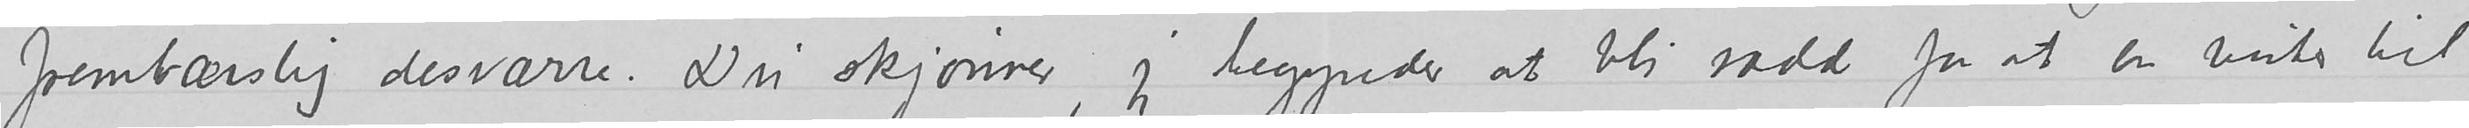frembærslig desværre.  Du skjønner, jeg begynder at bli rædd for at en vinter til
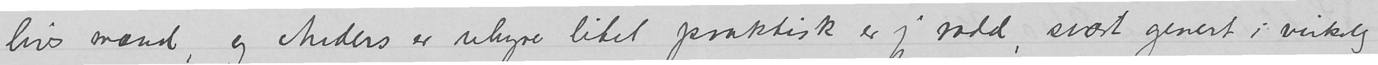livs mand, og Anders er uhyre litet praktisk er jeg rædd, svært genert i virkelig
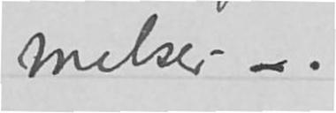melser -
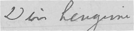Din hengivne
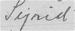Sigrid
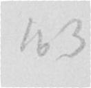163
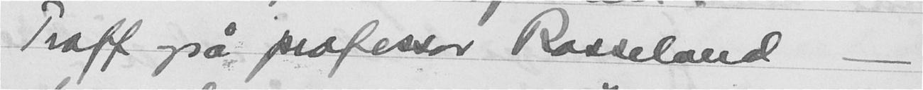Traff også professor Rasseland -
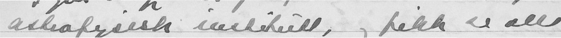astrofysisk institutt, og fikk se alle
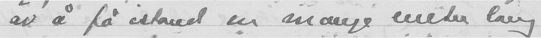av å få istand en mange meter lang
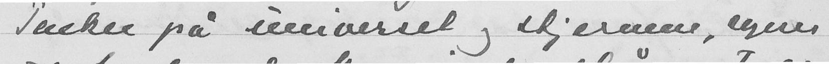Tenker på universet og stjernene, syner
